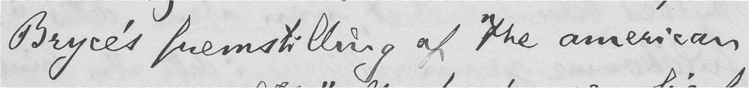Bryce's fremstilling af The american
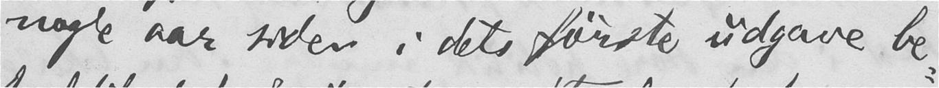nogle aar siden i dets første udgave be-
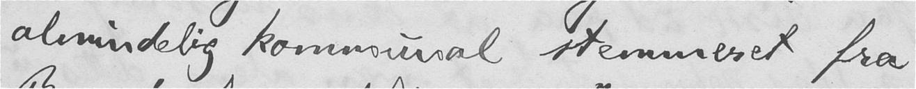almindelig kommunal stemmeret fra
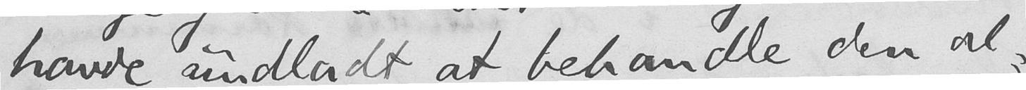havde undladt at behandle den al-
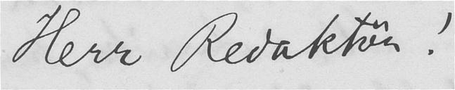Herr Redaktør!
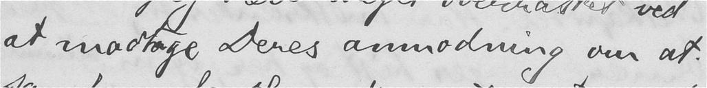at modtage Deres anmodning om at
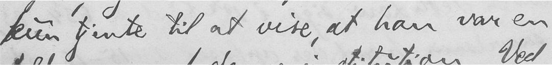kun tjente til at vise, at han var en
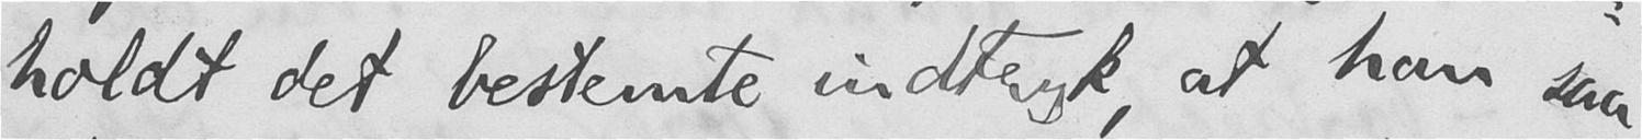holdt det bestemte indtryk, at han saa
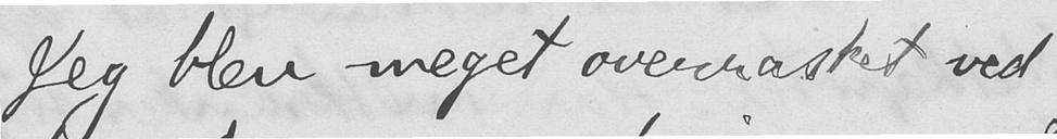Jeg blev meget overrasket ved
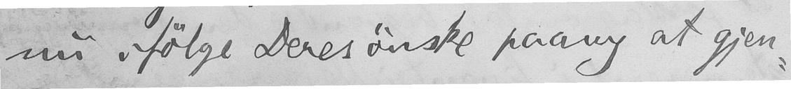nu ifølge Deres ønske paany at gjen-
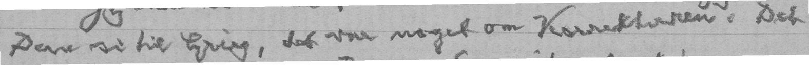Dem si til Grieg, det var noget om Korrekturen. Det
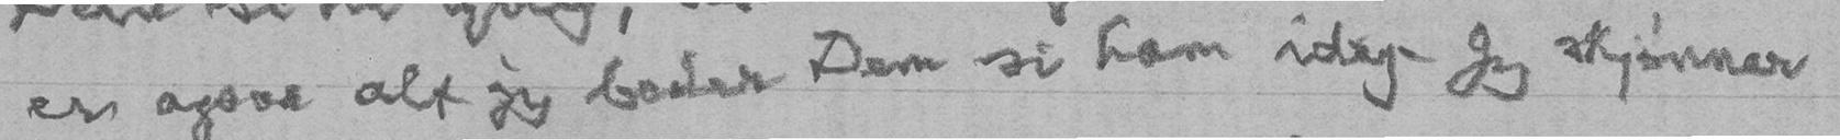er ogsaa alt jeg beder Dem si ham idag. Jeg skjønner
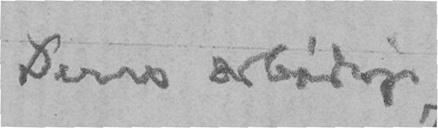Deres ærbødige
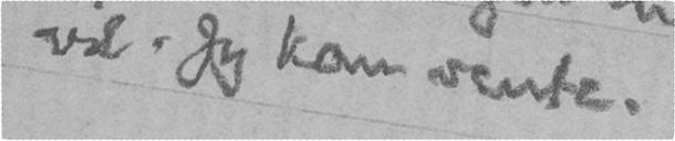vil. Jeg kan vente.
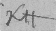KH
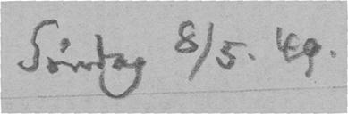Søndag 8/5. 49.
